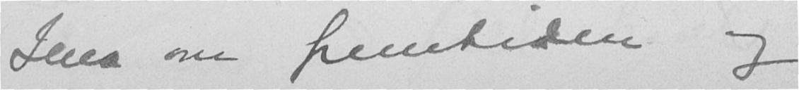Lena om fremtiden og
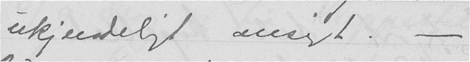ukjendeligt ansigt. -
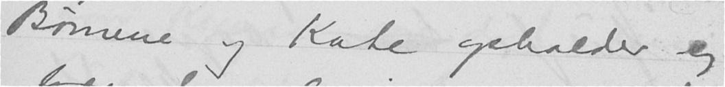Børnene og Kate opholder sig
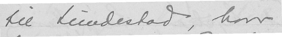til Lundestad, hvor
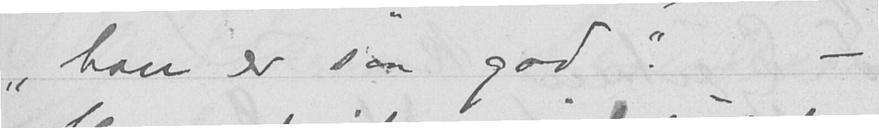"han er saa god". -
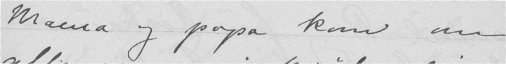Mama og papa kom om
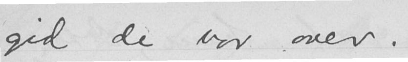gid de var over.
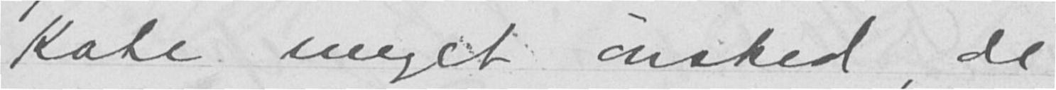Kate meget ønsked, de
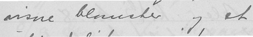visne blomster og et
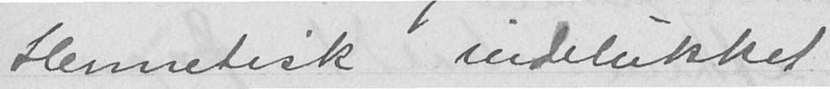Hermetisk indelukket
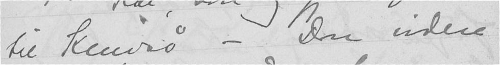til Knivsø - Don videre
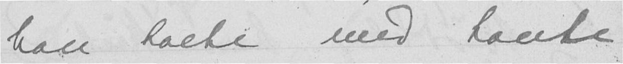han talte med tante
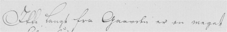Ikke langt fra Gaarden er en meget
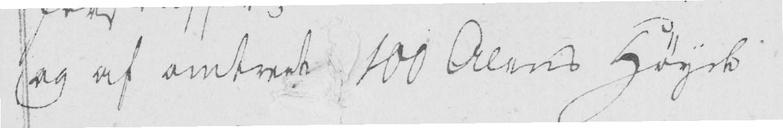og af omtrent 100 Alens Høyde.
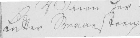better Smaaesteen
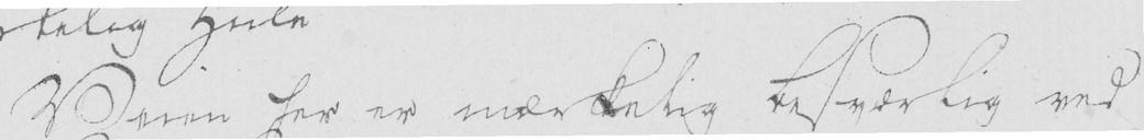Veien her er mærkelig besværlig ved
